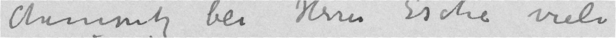Chemnitz bei Herrn Esche viele
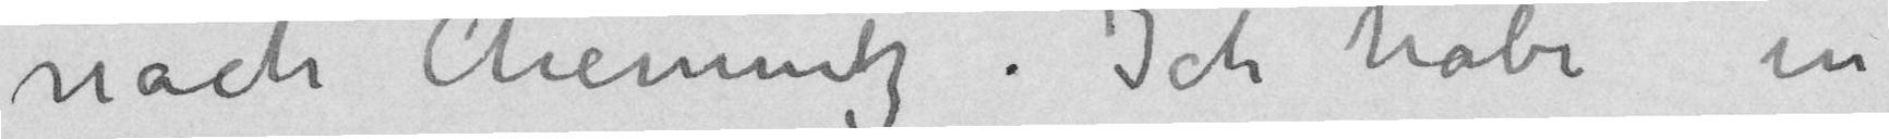nach Chemnitz. Ich habe in
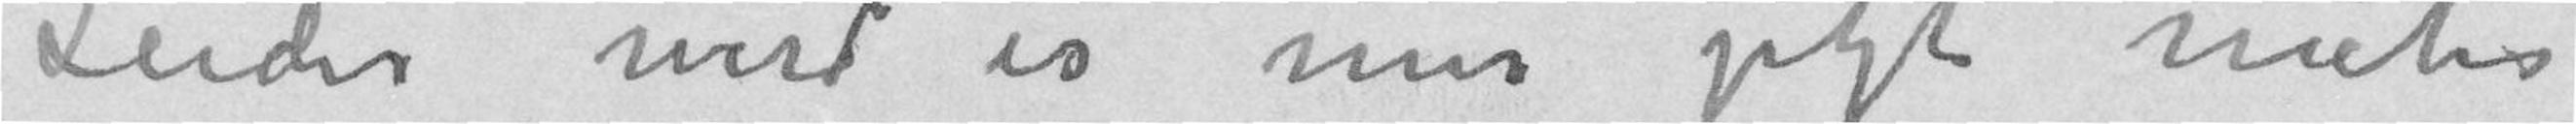Leider wird es mir jetzt mehr
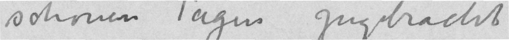schönen Tagen zugebracht
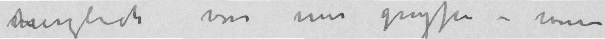herzlich von mir gruzsen – wenn
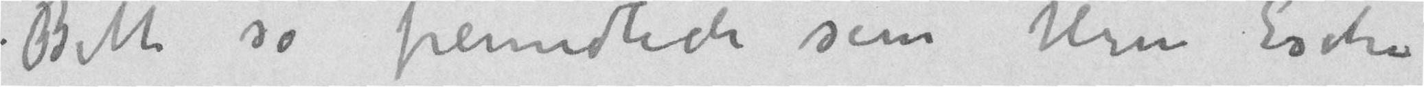Bitte so freundlich sein Hrn Esche
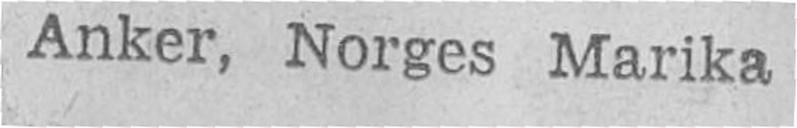Anker, Norges Marika
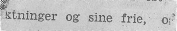ktninger og sine frie, o
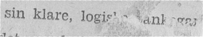sin klare, logis!  ank
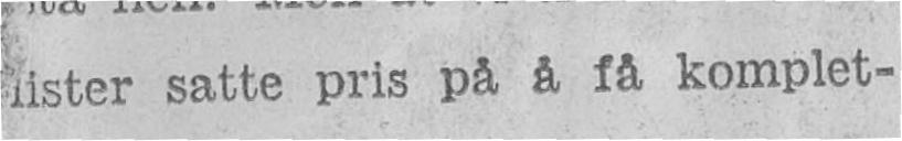lister satte pris på å få komplet-
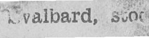valbard, stor
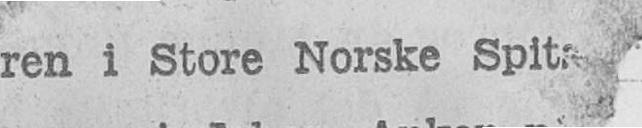ren i Store Norske Spit
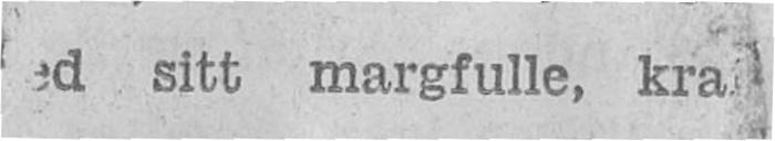ed sitt margfulle, kra
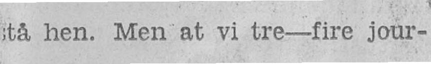tå hen. Men at vi tre—fire jour-
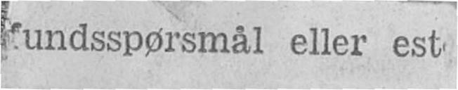fundsspørsmal eller est
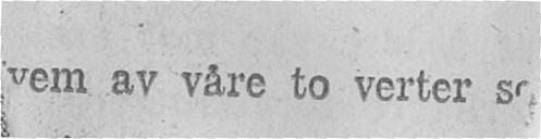vem av våre to verter s
